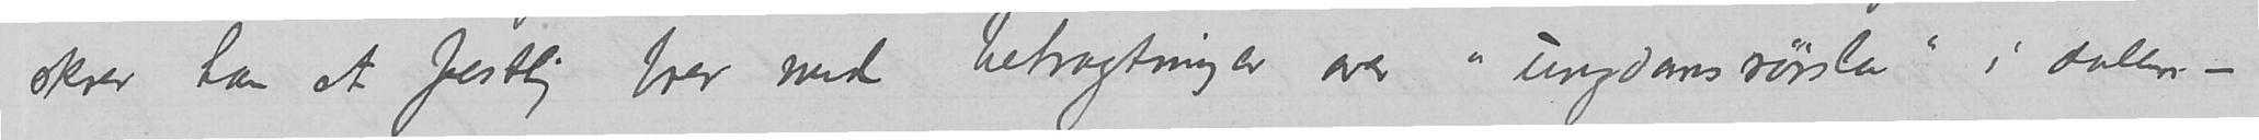skrev han et festlig brev med betragtninger over «ungdomsrørsle» i dalen -
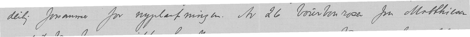deilig forsommer for nyplantingen. Av 26 bourbonroser fra Matthiesen
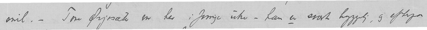snil. – Tore Ørjaseter var her i forrige uke – han er svært hyggelig, og efterpaa
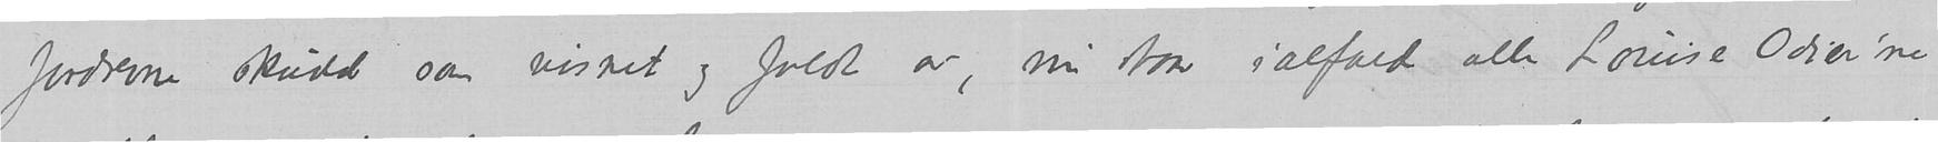fordrevne skudd som visnet og faldt av, nu staar ialfald alle Louise Odier’ne
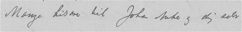Mange hilsener til Johan Anker og dig selv
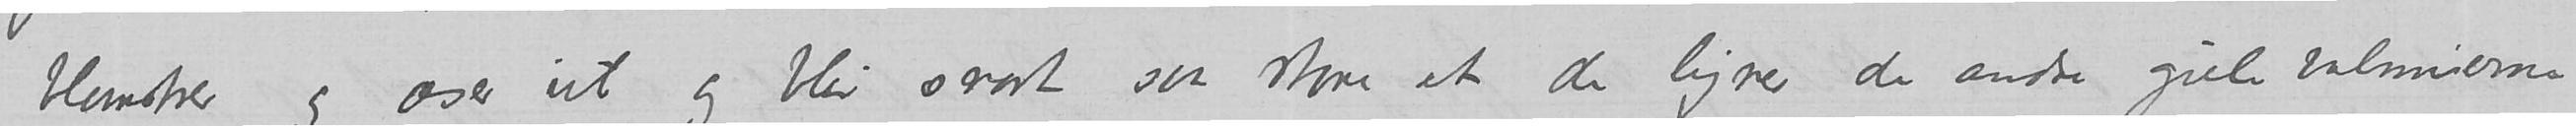blomstrer og oser ut og blir snart saa store at de ligner de andre gule valmuerne
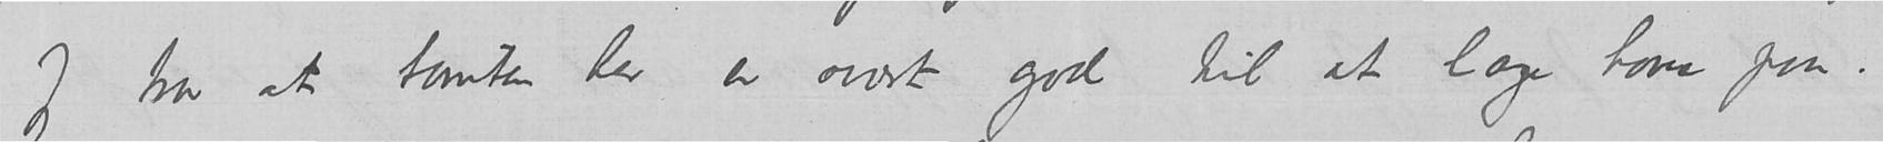Jeg tror at tomten her er svært god til at lage have paa!
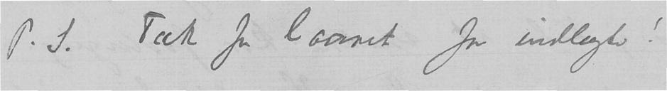P.S. Tak for laanet for indlagte!
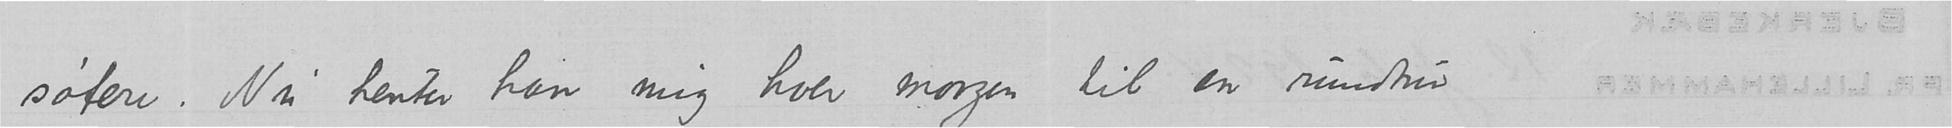søtere. Nu henter han mig hver morgen til en rundtur
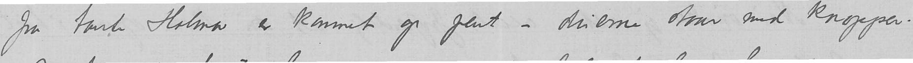fra tante Halma er kommet op pent – druerne staar med knopper.
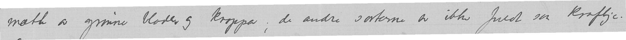mætte av grønne blader og knopper; de andre sorterne er ikke fuldt saa krafige.
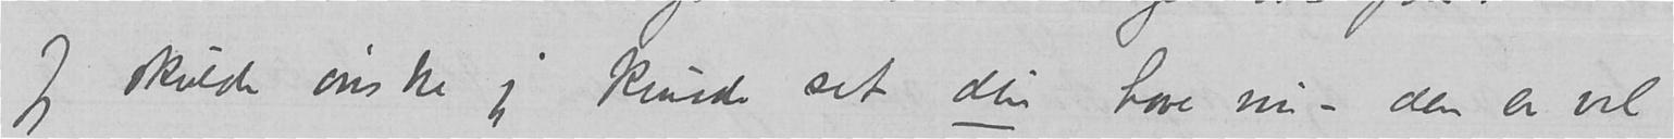Jeg skulde ønske jeg kunde set din have nu – den er vel
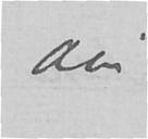din
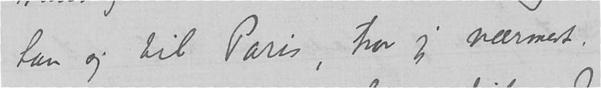han sig til Paris, tror jeg nærmest.
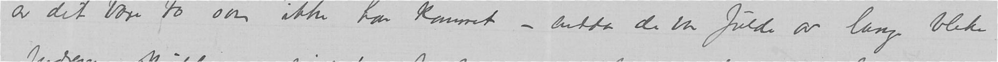er det bare to som ikke har kommet – endda de var fulde av lange bleke
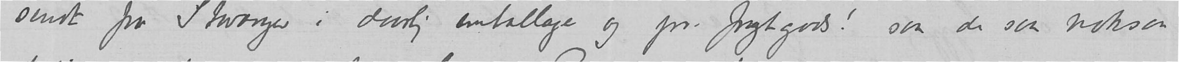sindt fra Stavanger i daarlig emballage og pr. frugtgods! som de saa noksaa
